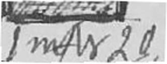1 mtr 20
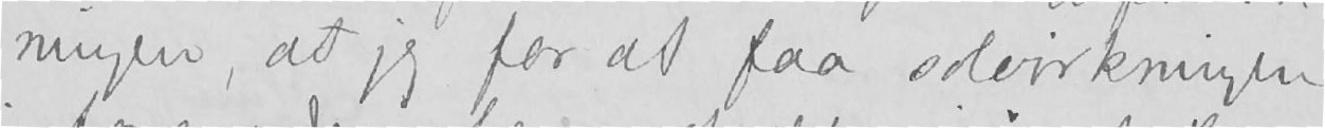ningen, at jeg for at faa solvirkningen
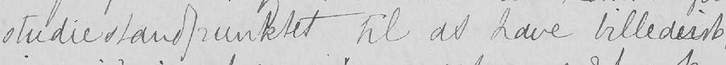studiestandpunktet til at have billedvirk-
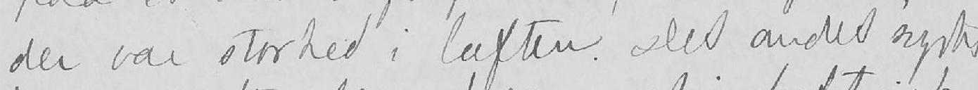der var storhed i luften. Det andet syntes
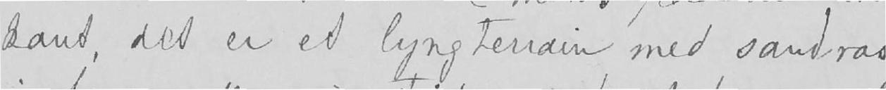kant, det er et lyngterrain med sandras
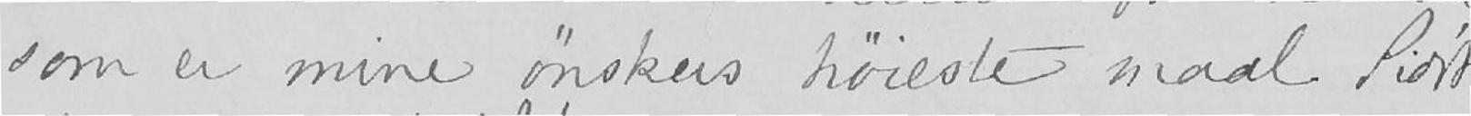som er mine ønskers høiste maal. Sidst
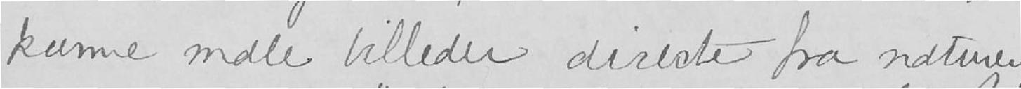kunne male billeder directe fra naturen,
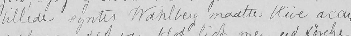billede syntes Wahlberg maatte blive accu-
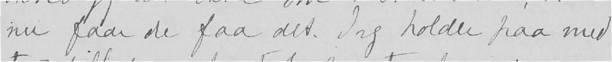nu faar de faa det. Jeg holder paa med
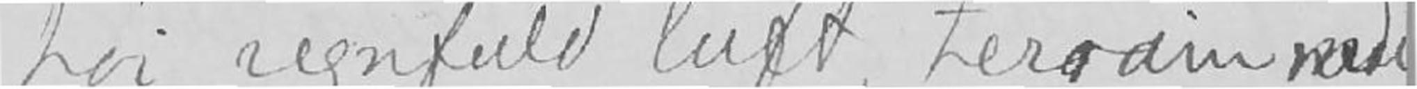høi regnfuld luft, terrain nede
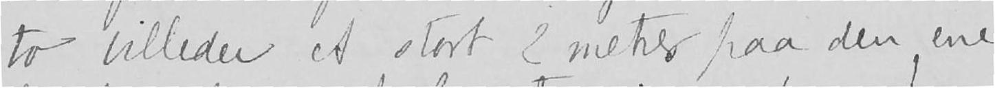to billeder et stort 2 metre paa den ene
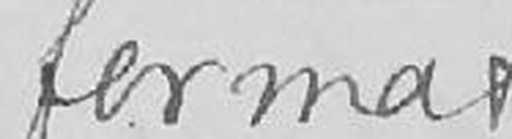format
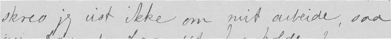skrev jeg vist ikke om mit arbeide, saa
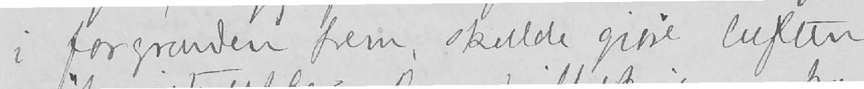i forgrunden frem, skulde giøre luften
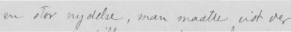en stor nydelse, man maatte vist der
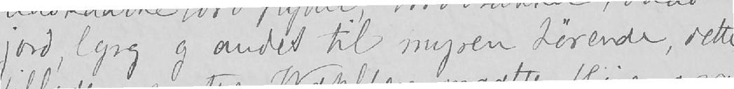jord, lyng og andet til myren hørende, dette
